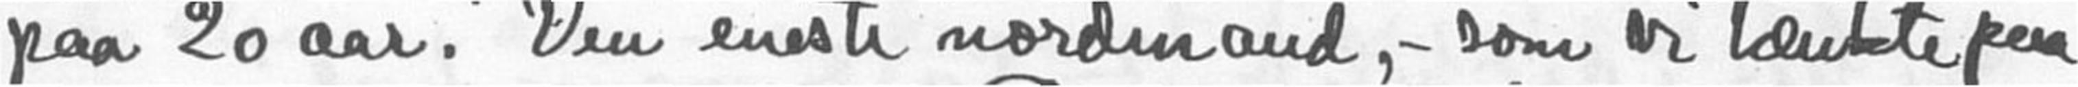paa 20 aar. Den eneste nordmand, - som vi tænkte paa
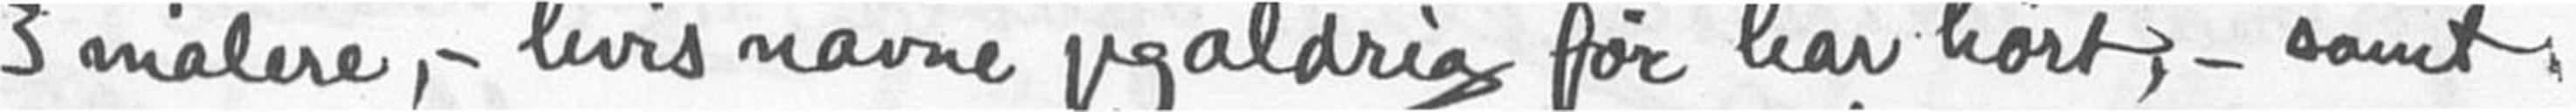3 malere, - hvis navne jeg aldrig for har hørt, - samt
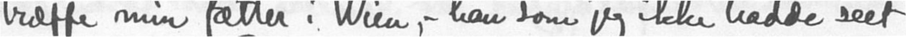træffe min fætter i Wien, - han som jeg ikke hadde seet
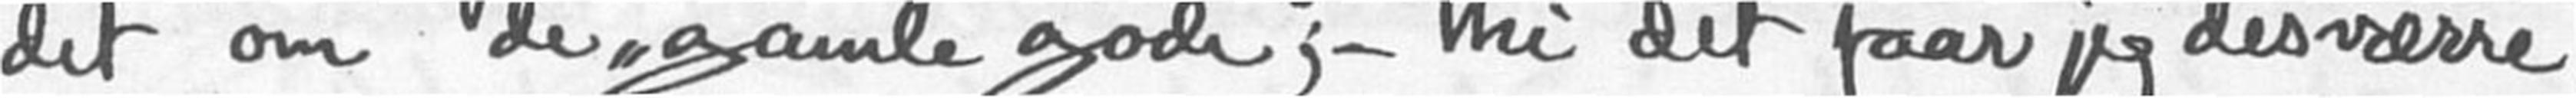det om de "gamle gode"; - thi det faar jeg desværre
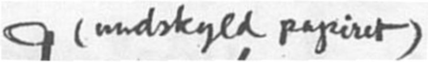(undskyld papiret)
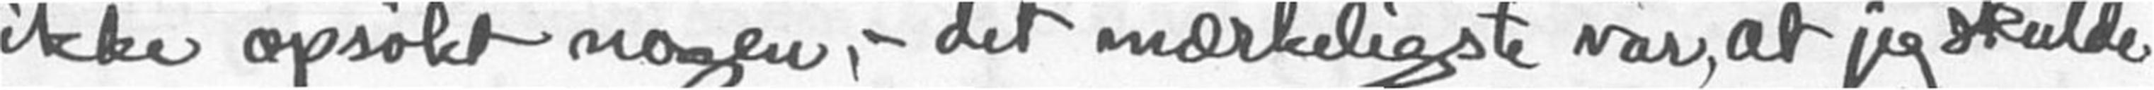ikke opsøkt nogen, - det mærkeligste var, at jeg skulde
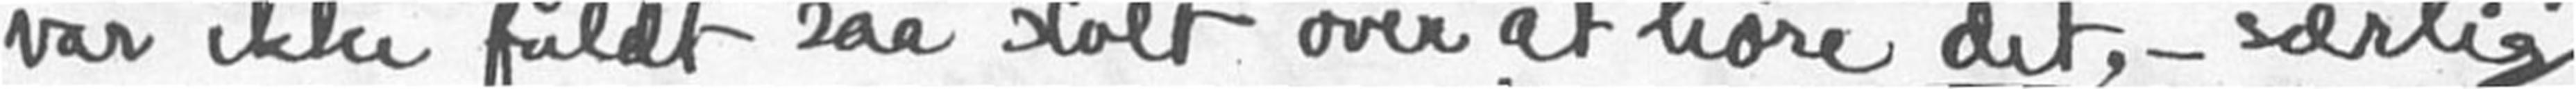var ikke fuldt saa stolt over at høre det, - særlig
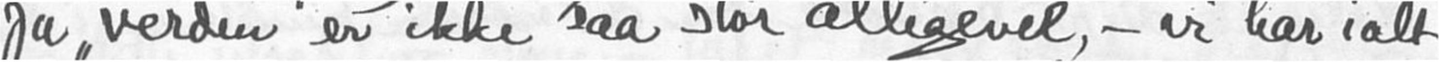Ja "verden er ikke saa stor" alligevel, - vi har ialt
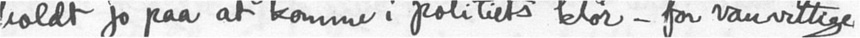holdt jo paa at komme i politiets klør - for vanvittige
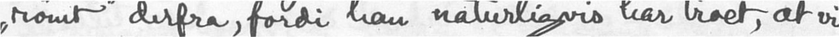"rømt" derfra, fordi han naturligvis har troet, at vi
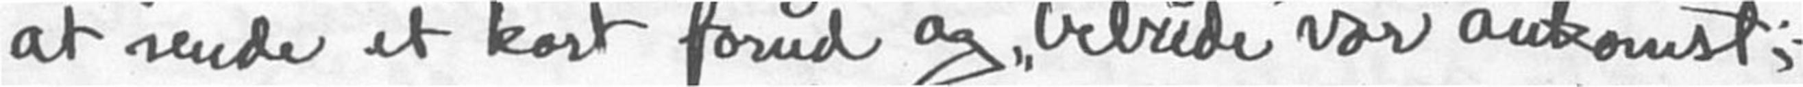at sende et kort forud og "bebude" vor ankomst; -
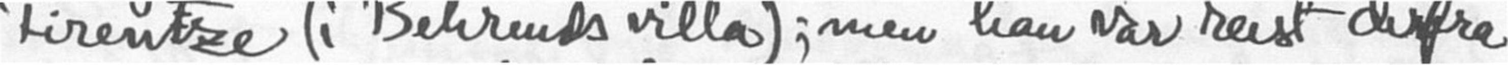Firenze (i Behrends villa); men han var reist derfra
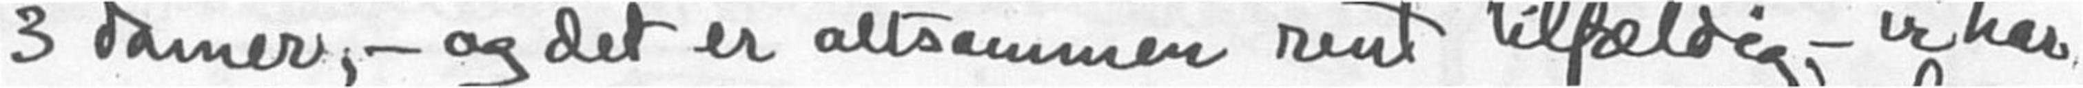3 damer, - og det er altsammen rent tilfældig,- vi har
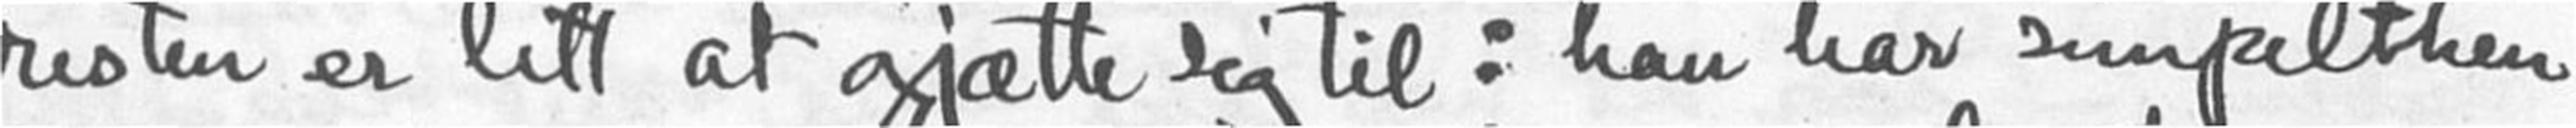resten er lett at gjætte sig til: han har simpelthen
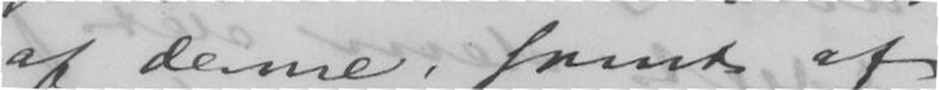af denne, samt af
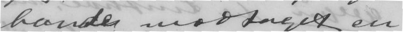havde modtaget en
